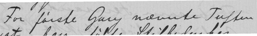For første Gang nævnte Tuften
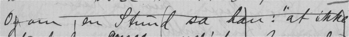Og om en Stund sa han: "at ikke
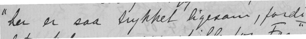"her er saa trykket ligesom, fordi
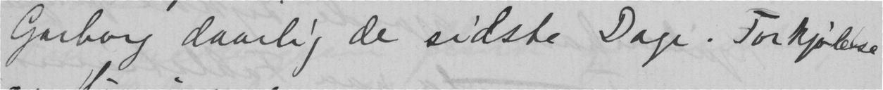Garborg daarlig de sidste Dage. Forkjølese
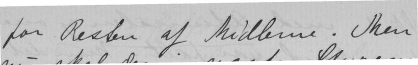for Resten af Midlerne. Men
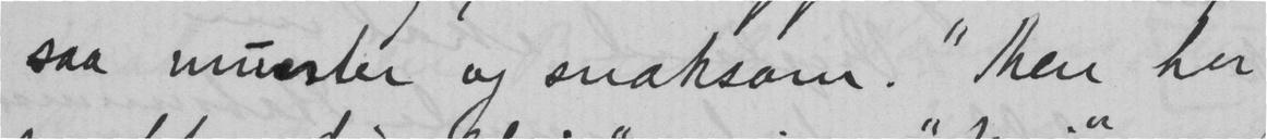saa munter og snaksom. "Men her
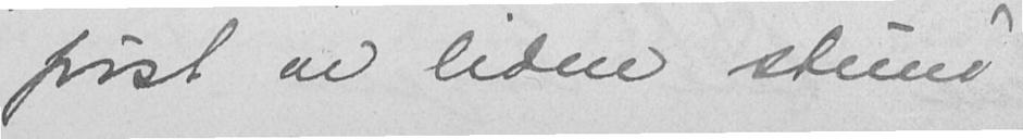først en liden stund
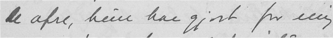de ofre, hun har gjort for mig
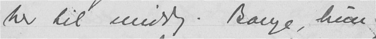her til middag. Bange, hun
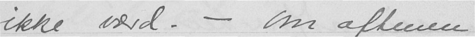ikke værd. - Om aftenen
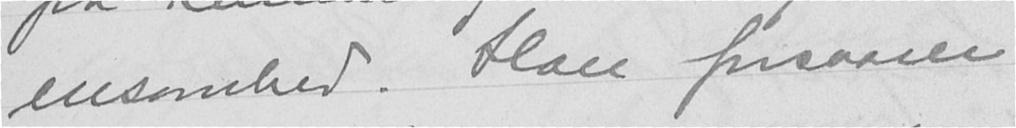ensomhed. Han forsvarer
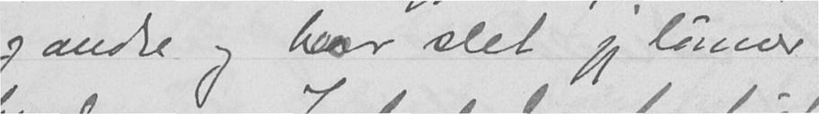og andre og hvor slet jeg lønner
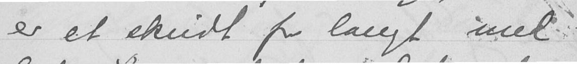er et skridt for langt med
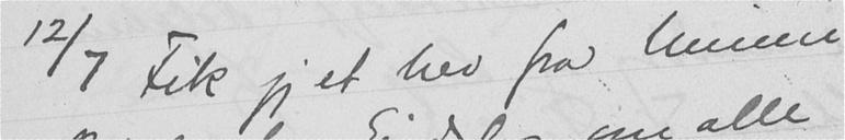12/7 Fik jeg et brev fra Minni
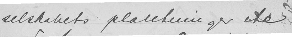selskabets plantninger etc
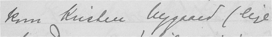kom Kristen Nygaard (lige
